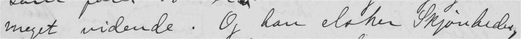meget vidende. Og han elsker Skjønheden,
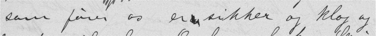som fører os er  sikker og klog og
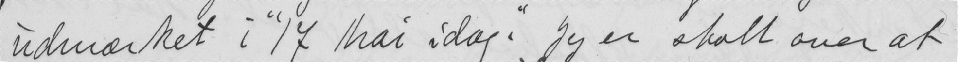udmærket i "17 Mai idag". Jeg er stolt over at
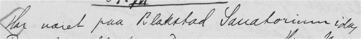Har været paa Blakstad Sanatorium idag
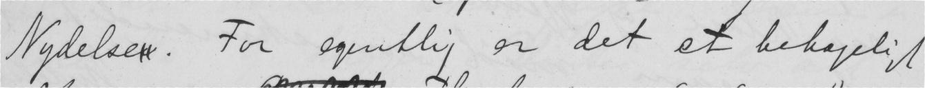Nydelse. For egentlig er det et behageligt
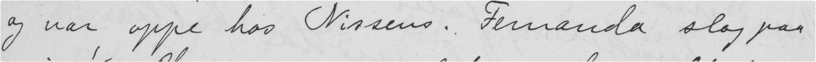og var oppe hos Nissens. Fernanda slog paa
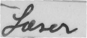Læser
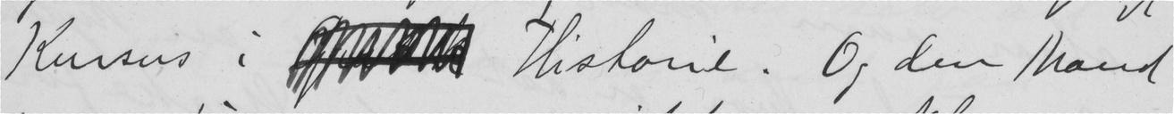Kurus i  Historie. Og den Mand
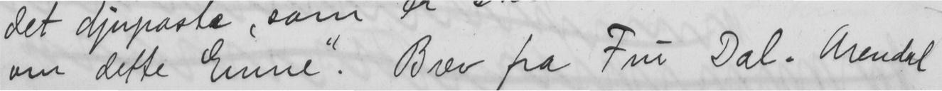om dette Emne. Brev fra Fru Dal. Arendal
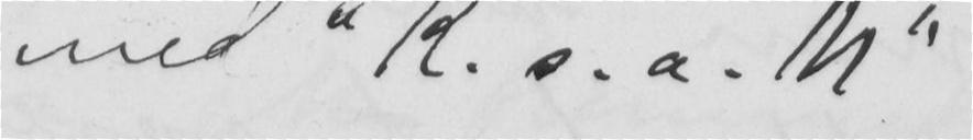med "K. s. a. M.",
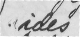ides
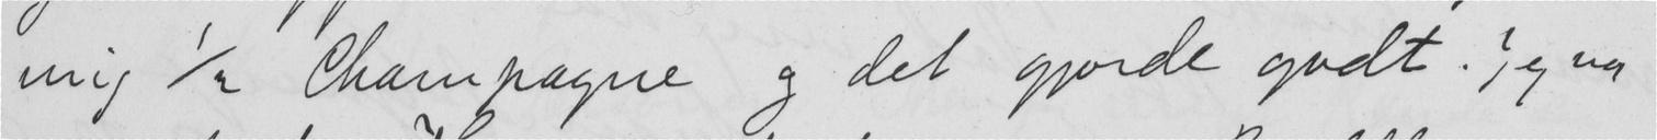mig 1/2 Champagne og det gjorde godt. Jeg var
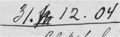31. 12.04
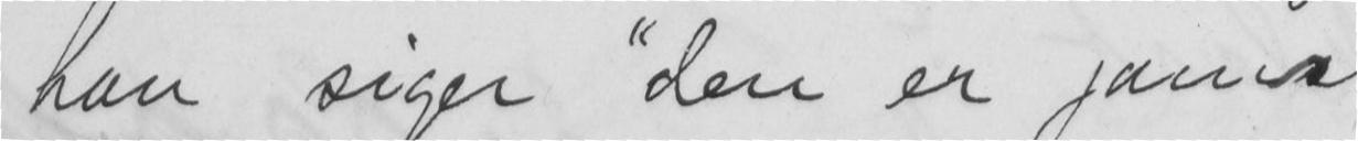han siger "den er jams
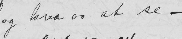og lærer os at se —
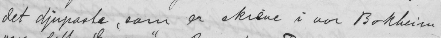det djupaste, som er skreve i vor Bokheim
